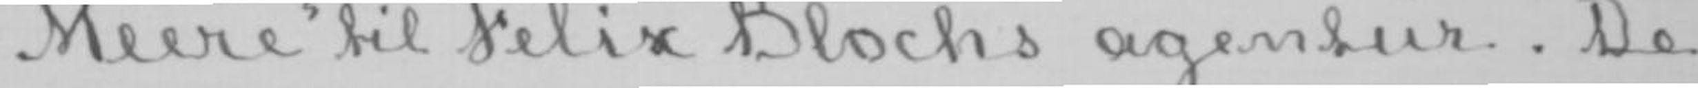Meere» til Felix Blochs agentur. De
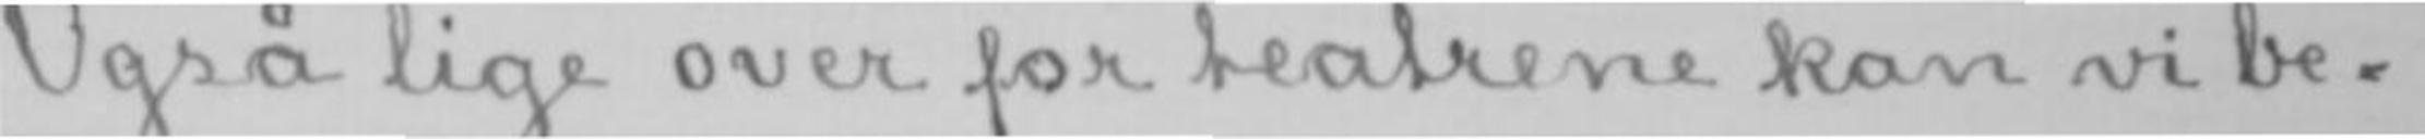Også lige over for teatrene kan vi be-
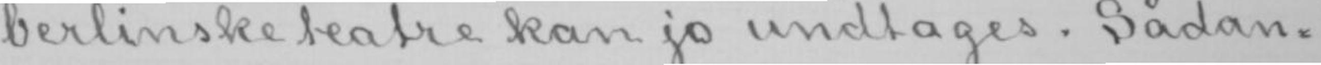berlinske teatre kan jo undtages. Sådan-
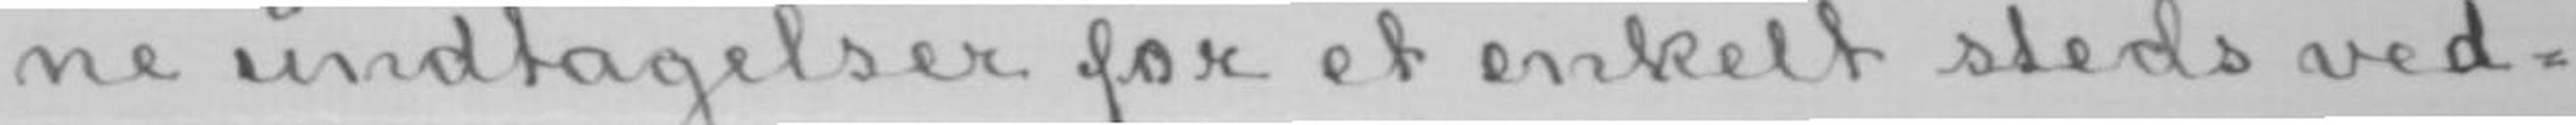ne undtagelser for et enkelt steds ved-
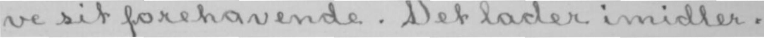ve sit forehavende. Det lader imidler-
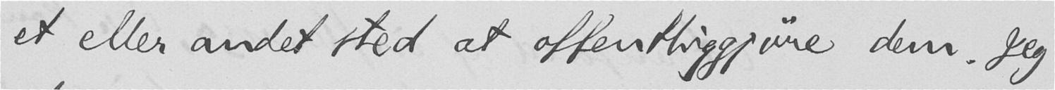et eller andet sted at offentliggjøre dem. Jeg
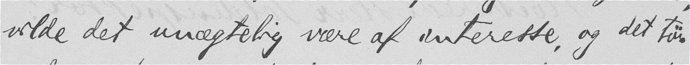vilde det unægtelig være af interesse, og det tør
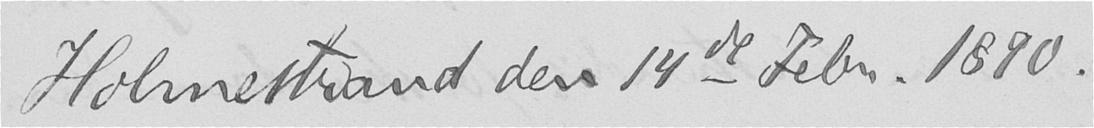Holmestrand den 14de Febr. 1890.
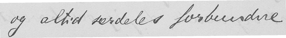og altid særdeles forbundne
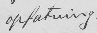opfatning.
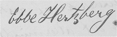Ebbe Hertzberg.
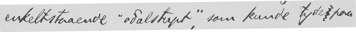enkeltstaaende "oðalstupt", som kunde tyde paa
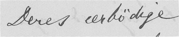Deres ærbødige
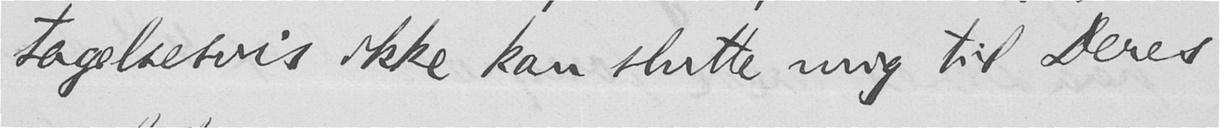tagelsesvis ikke kan slutte mig til Deres
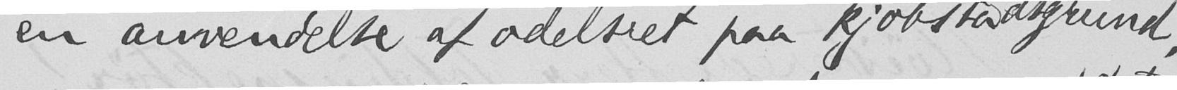en anvendelse af odelsret paa kjøbstadsgrund,
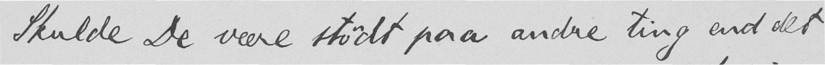Skulde De være stødt paa andre ting end det
